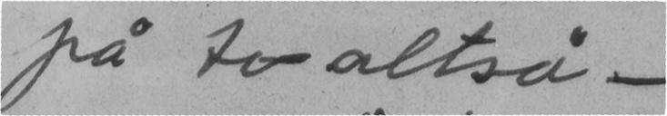på to altså -
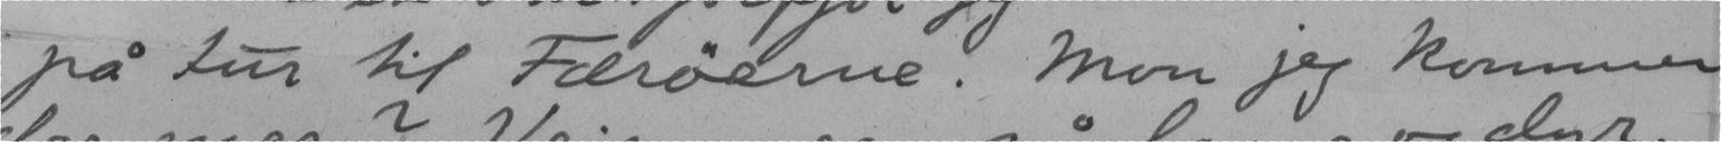på tur til Færøerne. Mon jeg kommer
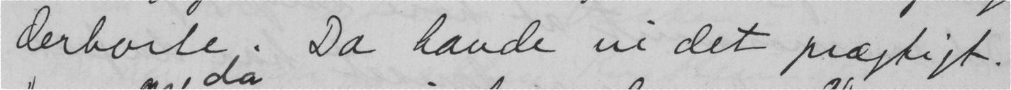derborte. Da havde vi det prægtigt.
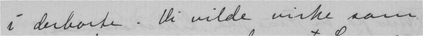i derborte. Vi vilde virke som
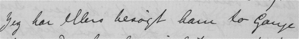Jeg har ellers besøgt ham to Gange
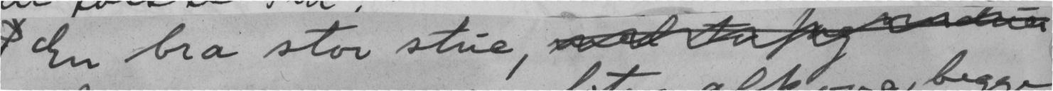En bra stor stue,
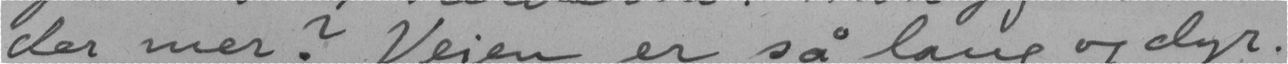der mer. Veien er så lang og dyr.
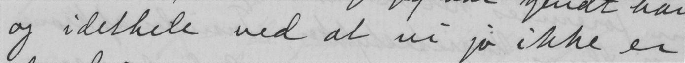og idethele ved at vi jo ikke er
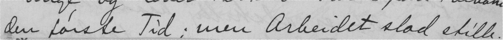den første Tid: men Arbeidet stod stille.
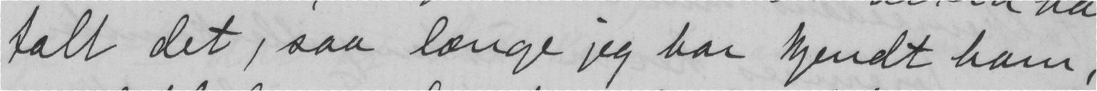talt det, saa længe jeg har kjendt ham,
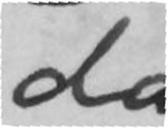da
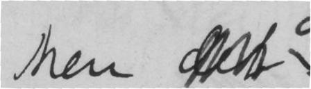Men
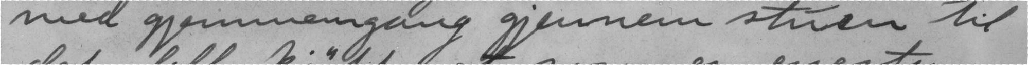med gjemmemgang gjennem stuen til
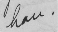han.
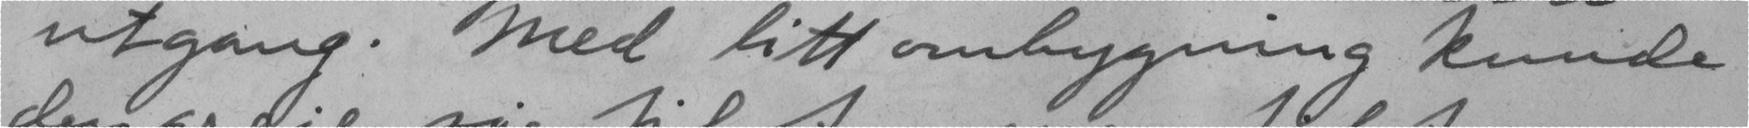utgang. Med litt ombygning kunde
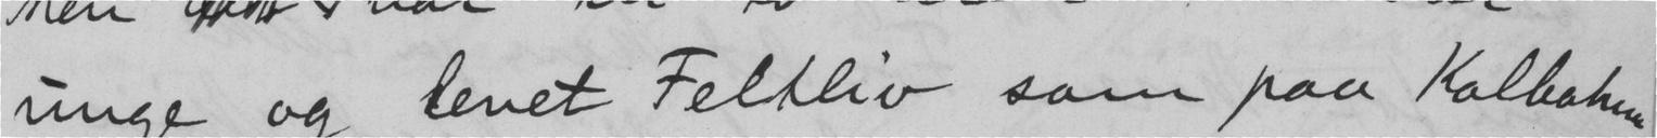unge og levet Feltliv som paa Kolboh\tn.
